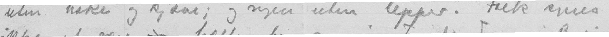uten hake og kjæve; og nogen uten lepper. Folk synes
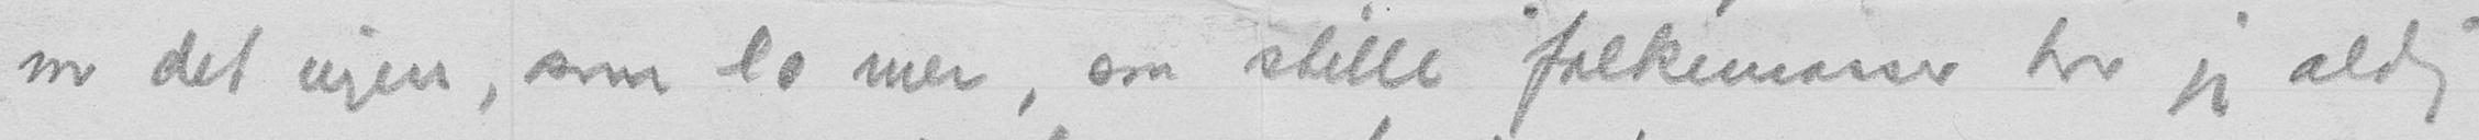var det ingen, som lo mer, saa stille folkemasser har jeg aldrig
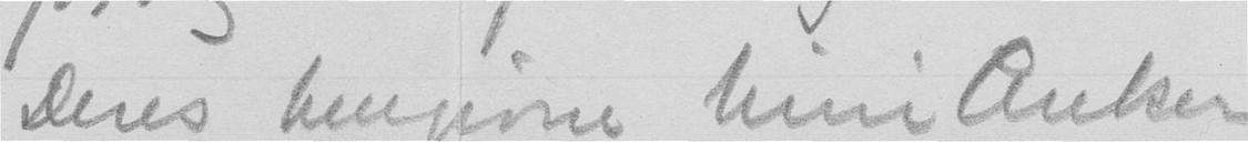Deres hengivne Nini Anker
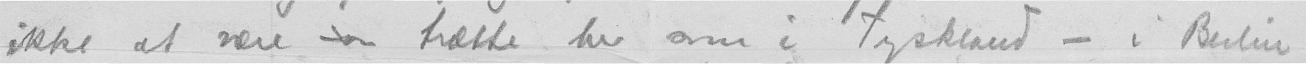ikke at være saa trætte her som i Tyskland - i Berlin
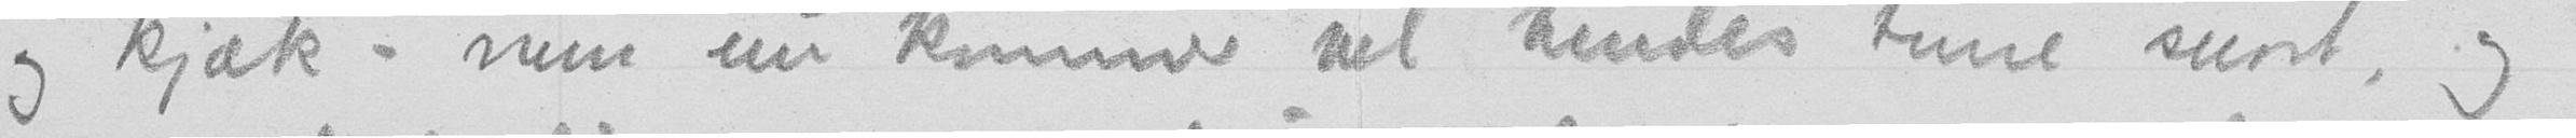og kjæk - men nu kommer vel hendes time snart, og
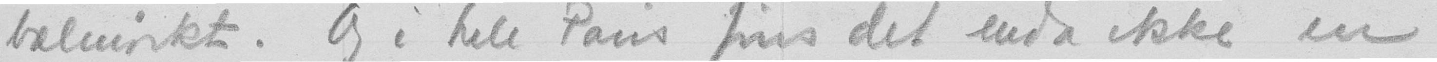bælmørkt. Og i hele Paris fins det enda ikke en
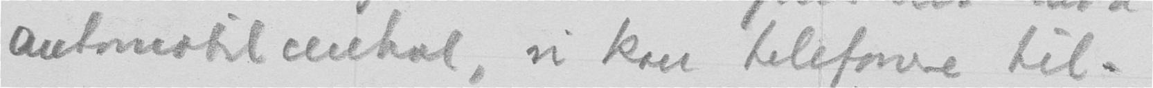automobilcentral, vi kan telefonere til.
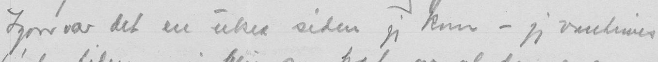Igaar var det en uke siden jeg kom - jeg vantrives
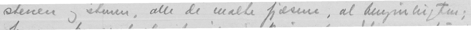stenen og stenen, alle de malte fjæsene, al hengihigten;
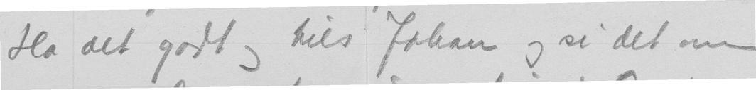Ha det godt og hils Johan og si det om
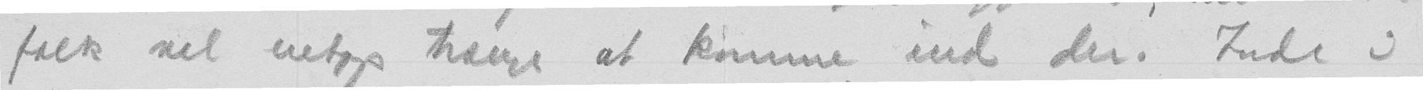folk vel nutop trænge at komme ind der. Inde i
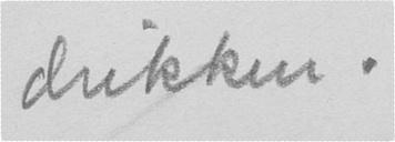drikken.
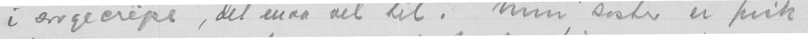i sørgecrêpe, det maa vel til. Min søster er frisk
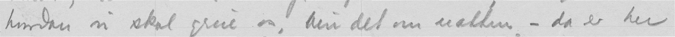hvordan vi skal greie os, blir det om natten - da er her
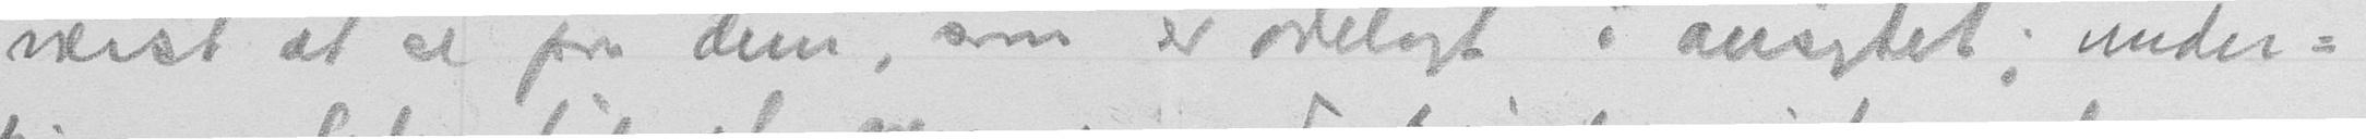værst at se paa dem, som er ødelagt i ansigtet; under-
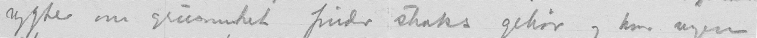rygter om grusomhet finder straks gehør og hvor nogen
